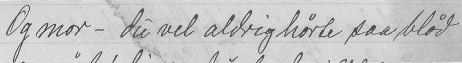Og mor - du vel aldrig hørte saa blød
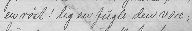en røst! lig en fugls den være;
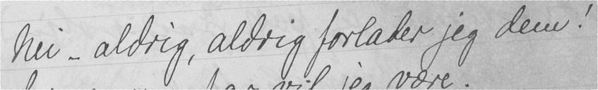Nei - aldrig, aldrig forlader jeg dem!
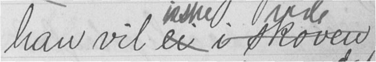han vil ei i skoven
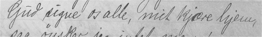Gud signe os alle, mit kjære hjem,
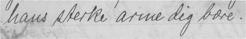hans sterke arme dig bære.
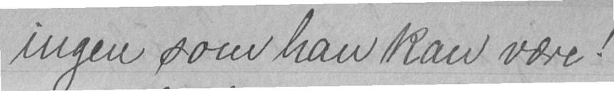ingen som han kan være!
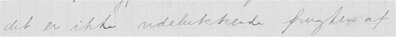det er ikke udelukkende frugten af
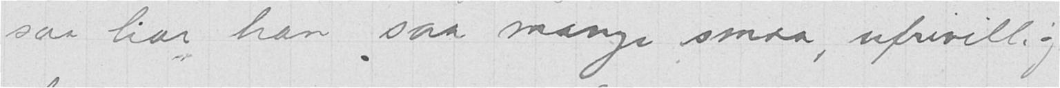saa har han saa mange smaa, ufrivillig
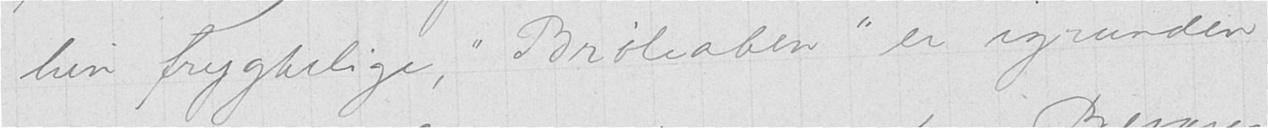hin frygtelige, "Brøleaben" er igrunden
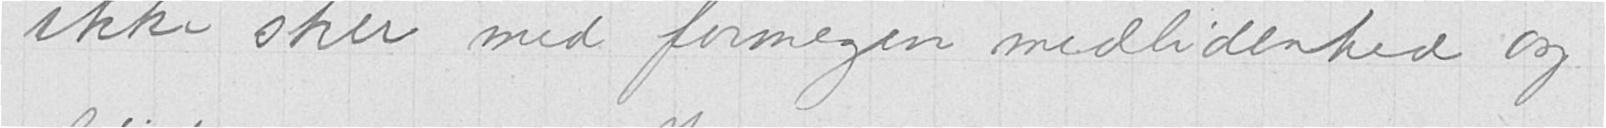ikke sker med formegen medlidenhed og
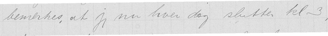bemerkes, at jeg nu hver dag slutter kl 3,
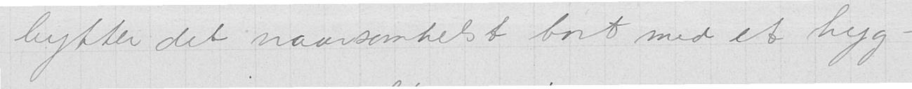bytter det naarsomhelst bort med et hyg-
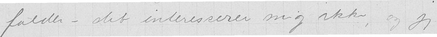falder - det interesserer mig ikke, og jeg
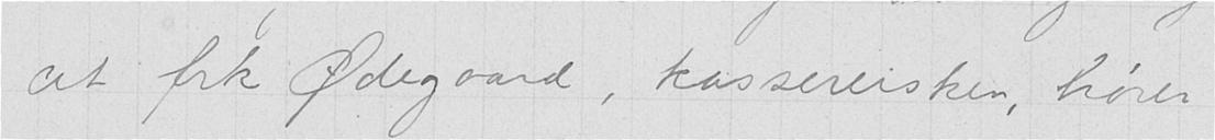at frk. Ødegaard, kasserersken, hører
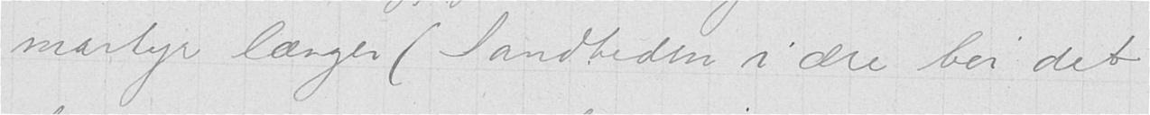martyr længer (Sandheden i ære bør det
